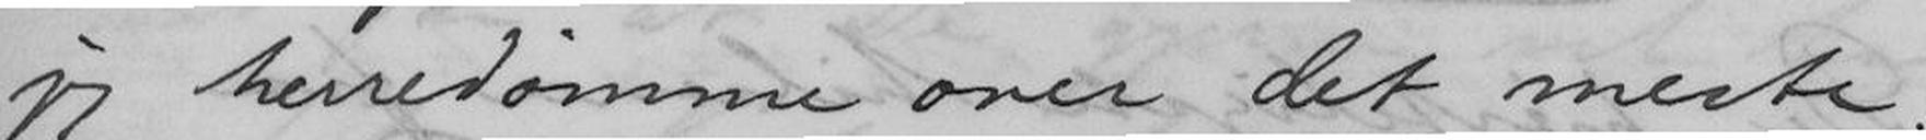jeg herredømme over det meste.
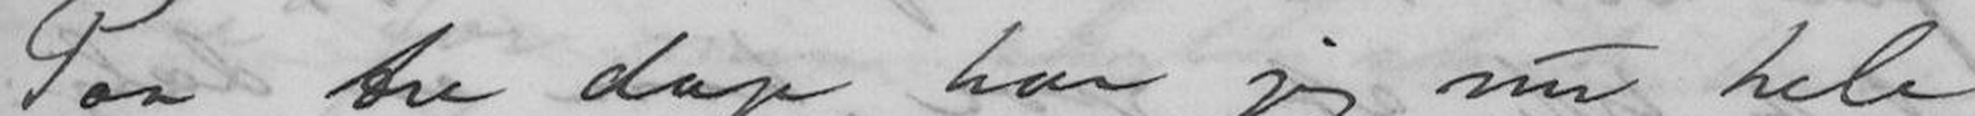Paa tre dage har jeg nu hele
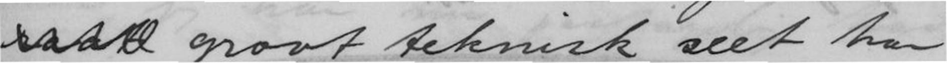grovt teknisk seet har
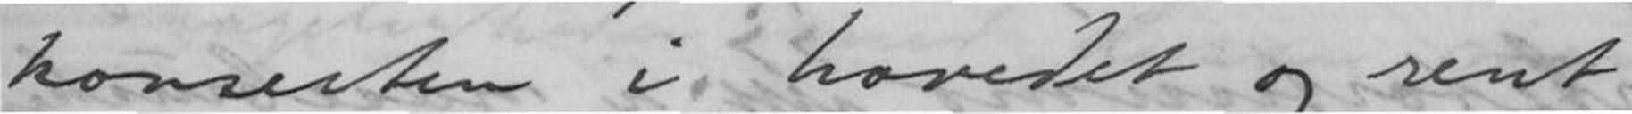konserten i hovedet og rent
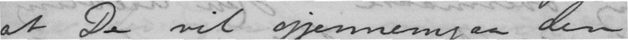at De vil gjennemgaa den
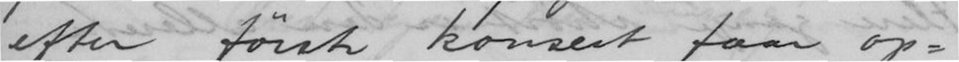efter første konsert faar op-
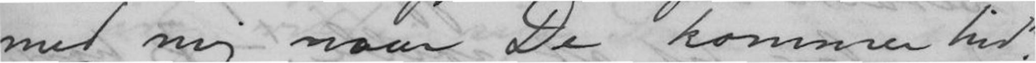med mig naar De kommer hid?
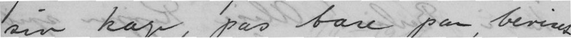sin kage, pas bare paa, beviset
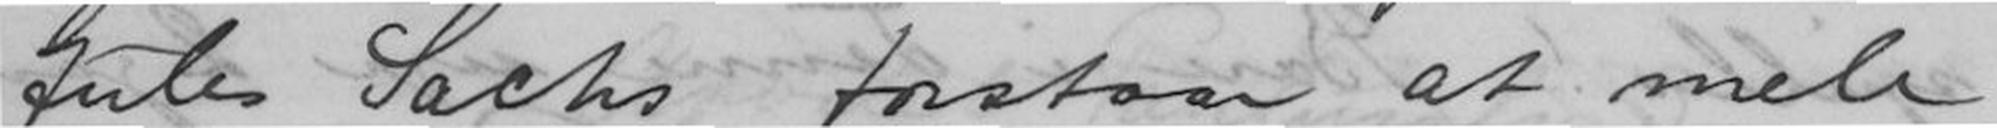Jules Sachs forstaar at mele
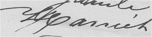Harriet
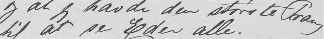og at jeg havde den største Trang
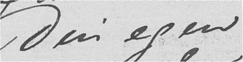Din egen
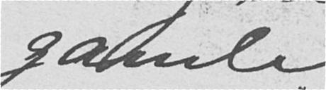gamle
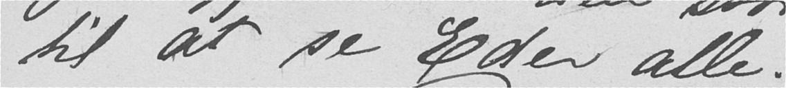til at se Eder alle.
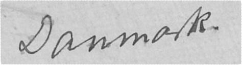Danmark.
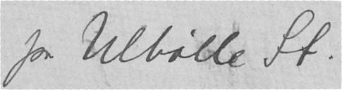pr Ulbølle St.
